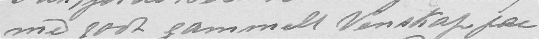med godt gammelt Venskap paa
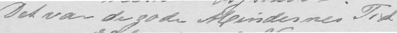Det var de gode Mindernes Tid
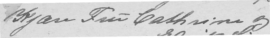Kjære Fru Cathrine og
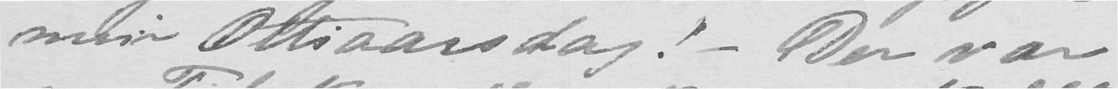min Ottiaarsdag! - Der var
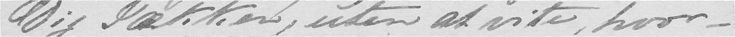Dig Jækken, uten at vite, hvor-
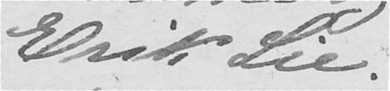Erik Lie.
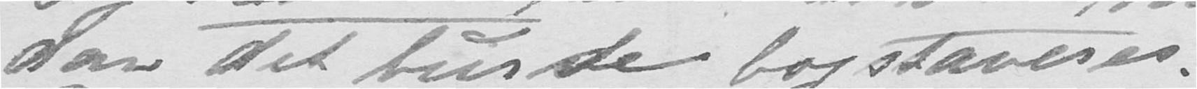dan det burde bogstaveres.
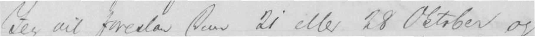Jeg vil foreslaa Den 21 eller 28 Oktober og
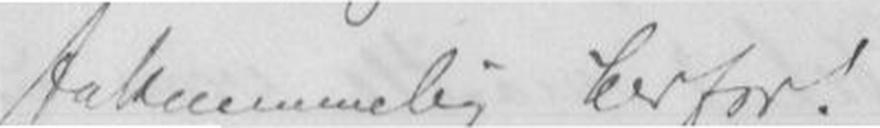taknemmlig herfor !
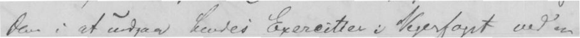Dem i at udgaa hevdes Exercitter i ved en
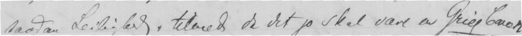saadan Leilighed, det jo skal være en Grieg Concert.
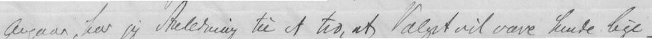angaar, har jeg Anledning til at tro, at Valget vil være ,
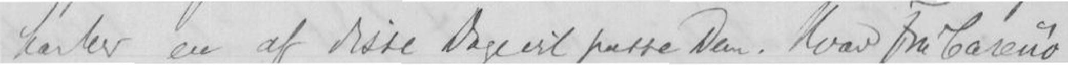tænker en af disse passse Dem. Hvad Fru Careno
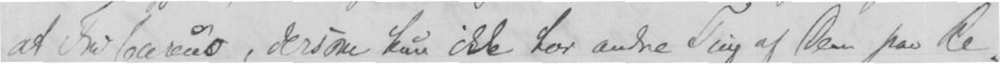at Fru Careno, dersom hun ikke for andre Ting af Dem paa Re-
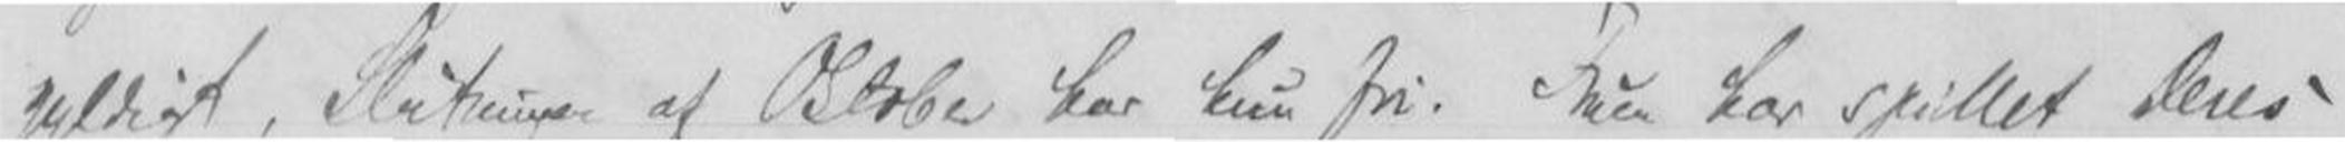gyldigt, af Oktobr. har haun fri. Fru Car. spillet Deres
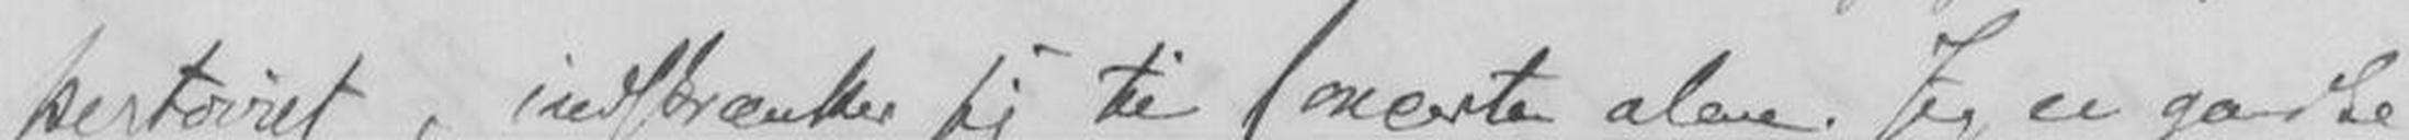pertoiret, jeg Concerten alene. Jeg er ganske
